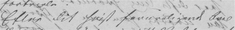Efter Dit høist foruroligende Brev
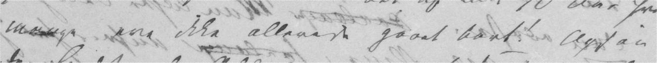mange ere ikke allerede gaaet bort! Ogsaa
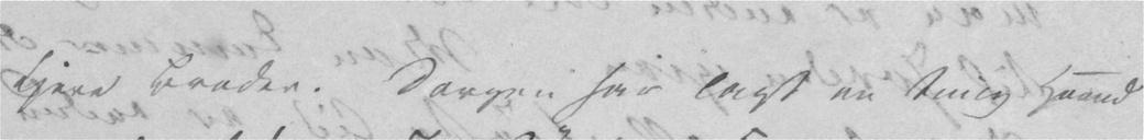Kjere Broder. Sorgen har lagt en tung Haand
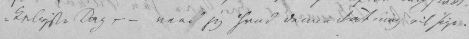skrækkeligste Dag – – veed jeg hvad denne Fatning vil sige.
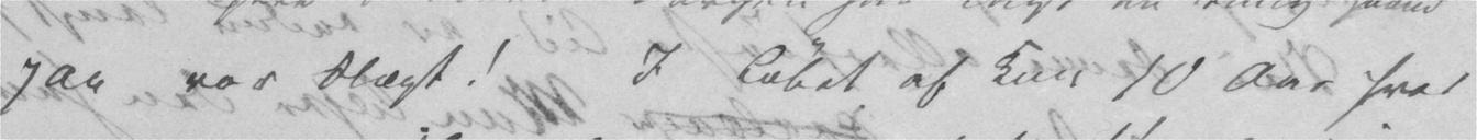paa vor Slægt! I Løbet af kun 10 Aar hvor
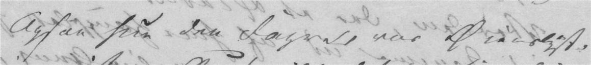Ogsaa hun den Fagre, vor Øienslyst,
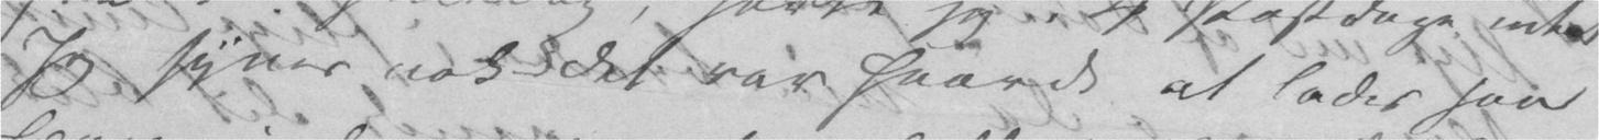Jeg synes nok det var haardt at lades saa
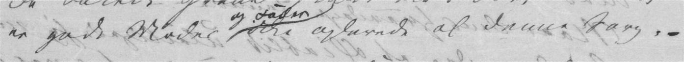er godt Moder og Fader ikke oplevede al denne Sorg. –
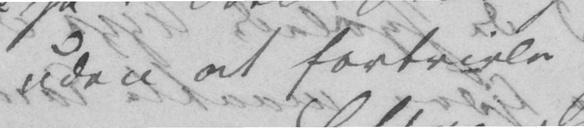uden at fortvivle.
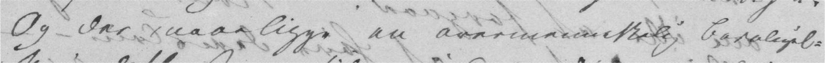Og der maae ligge en overmenneskelig Beroligel-
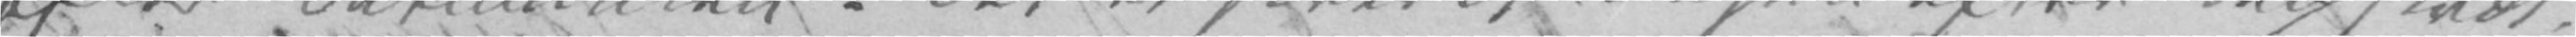efter Datummen – det er skrevet Dagen efter den næst
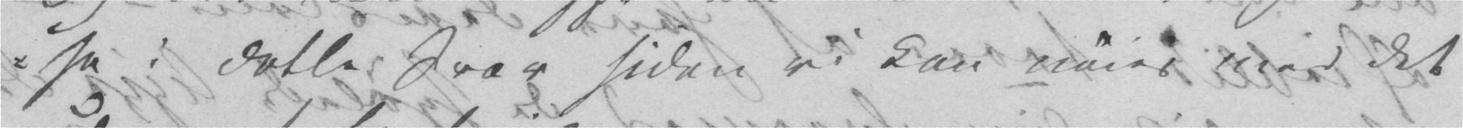se i dette Svar siden vi kan nøies med det
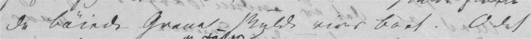de bøiede Grene – skulde rives bort. O det
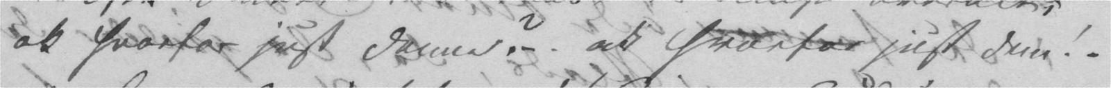ak hvorfor just denne?– ak hvorfor just dem! –
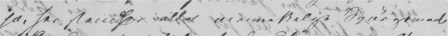ja, her standser alle menneskelige Spørgsmaal
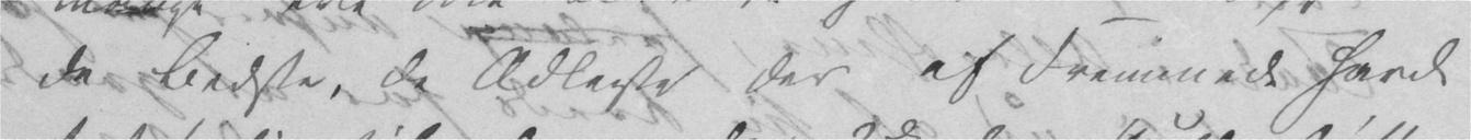de Bedste, de Ædleste der af Fremmede havde
